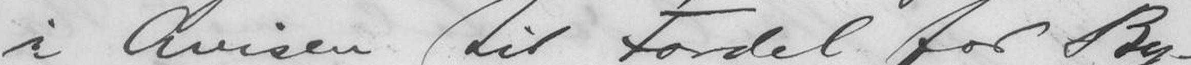i Avisen til Fordel for By-
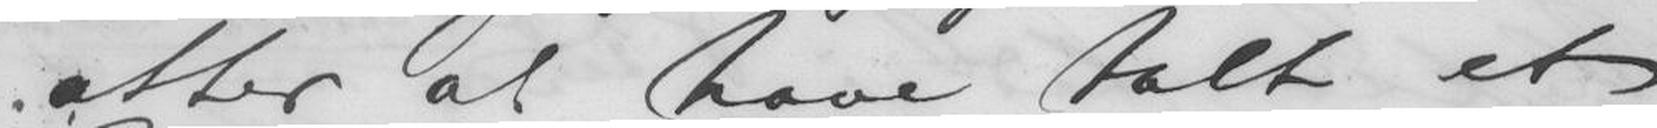atter at have talt et
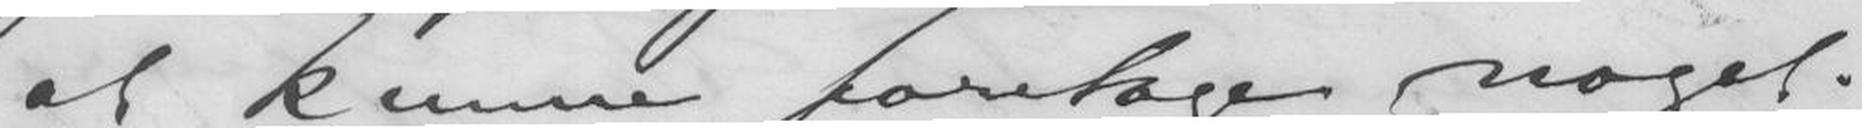at kunne foretage noget.
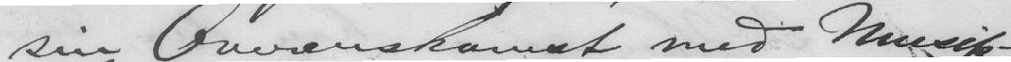sin Overenskomst med Musik-
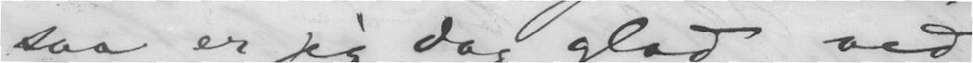saa er jeg dog glad ved
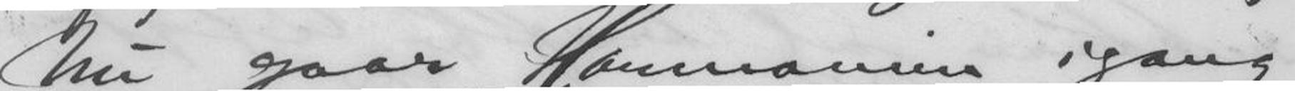Nu gaar Harmonien igang
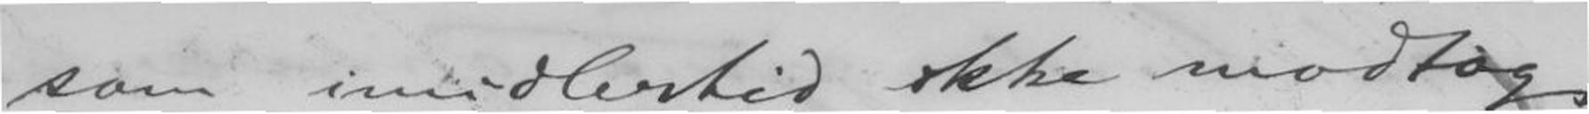som imidlertid ikke modtog
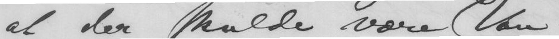at der skulde være Van-
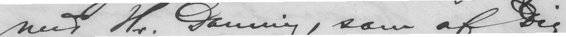med Hr. Danning, som af Dig
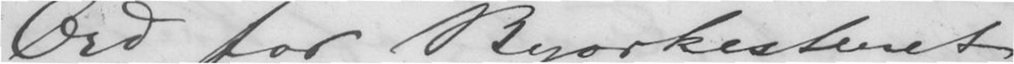Ord for Byorkesteret,
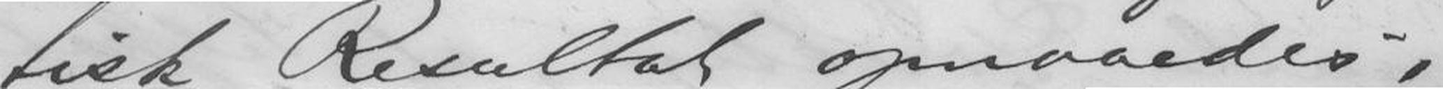tisk Resultat opnaaedes,
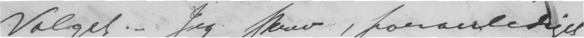Valget. Jeg skrev, foranlediget
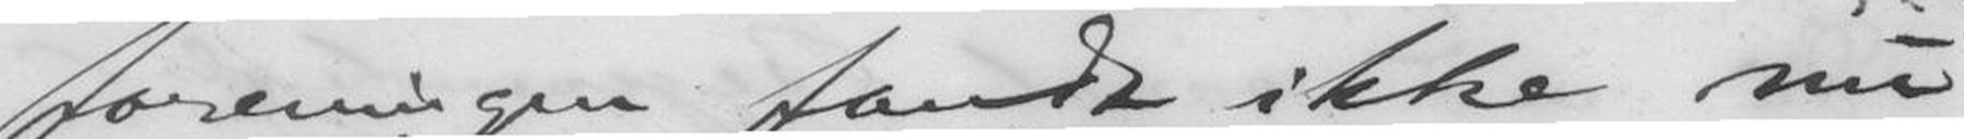foreningen fandt ikke nu
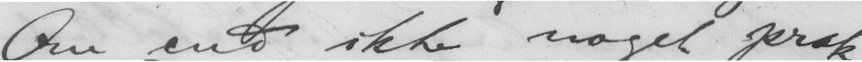Om end ikke noget prak-
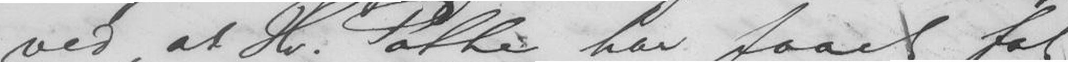ved, at Hr. Pottè har faaet fat
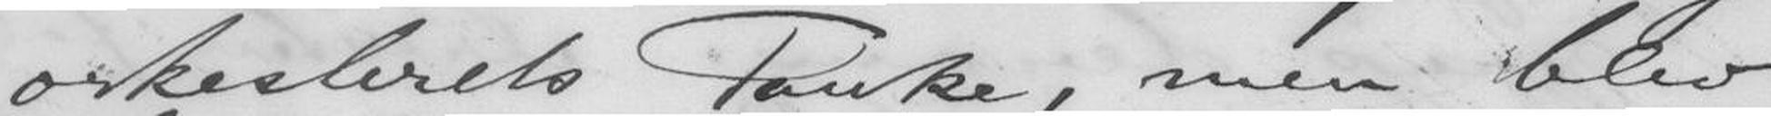orkesterets Tanke, men blev
